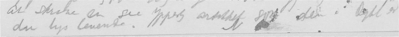at skrive en så ypperlig artikkel som den i Dagbl. er
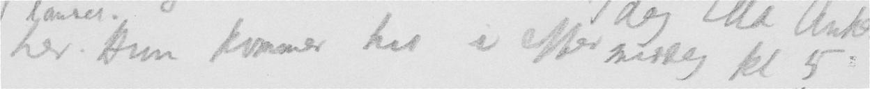her. Hun kommer hit i eftermiddag kl 5
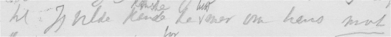til jeg vilde kende de mer om hans mot
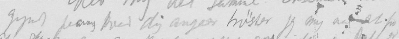opsyn heay hvad dig angår trøster jeg mig med at for
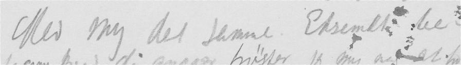Glld My det samme. Etserlte che?
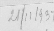21/11/1937
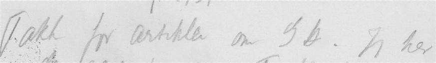Takk for artikler om G.H. Jeg har
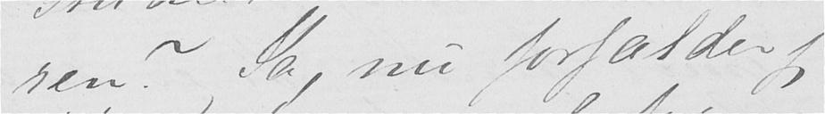ren? Ja, nu forfalder jeg
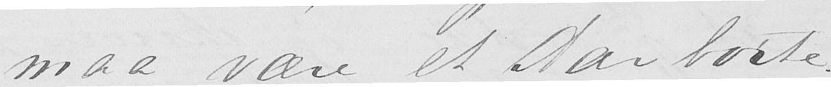maa være et Aar borte.
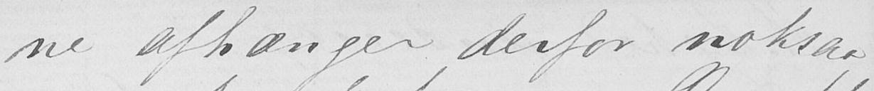ne afhænger derfor noksaa
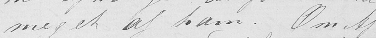meget af ham. Om Af-
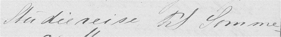Studiereise til Somme-
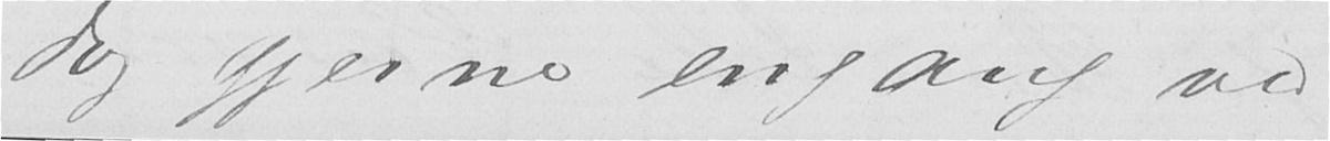dog gjerne engang ved
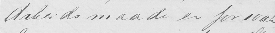Arbeidsmaade er forsvar-
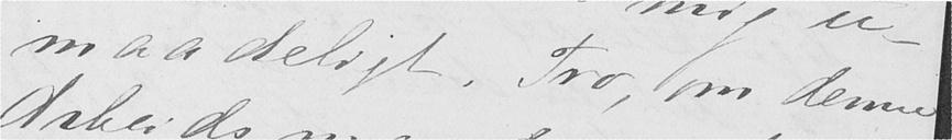maadeligt. Tro, som denne
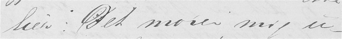lier. Det morer mig u-
